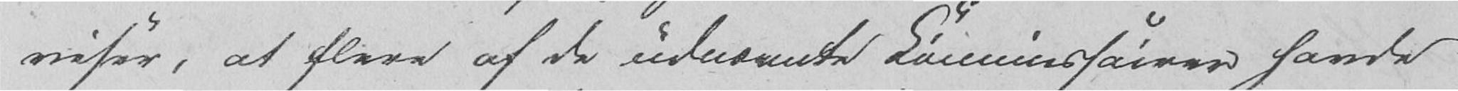viser, at flere af de udnævnte Kommisairer havde
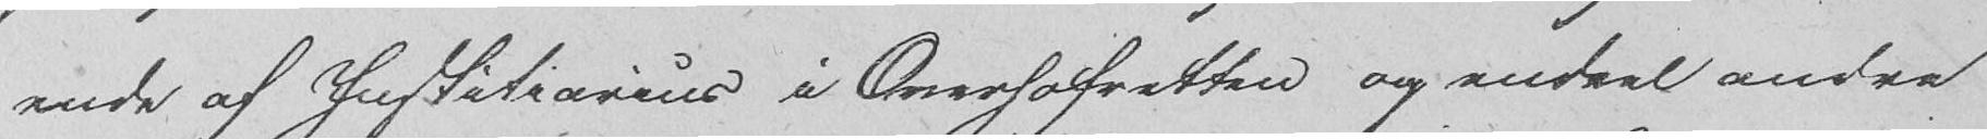ende af Justitiarius i Overhofretten og endeel andre
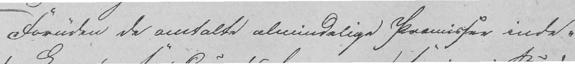Foruden de omtalte almindelige Premisser inde-
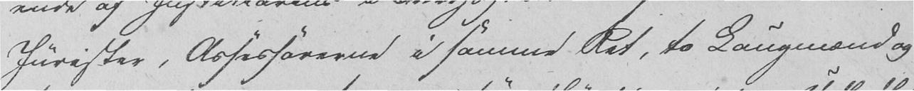Jurister, Assessorerne i samme Ret, to Laugmænd og
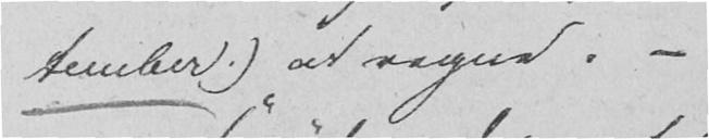tember.) at regne. -
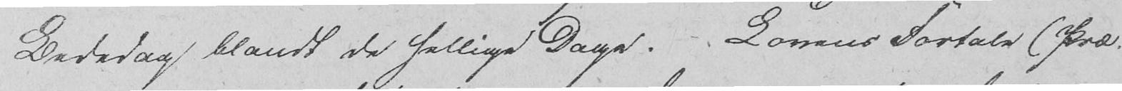Bededag blandt de hellige Dage. Lovens Fortale (Præ-
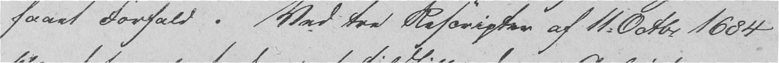faaet Forhold. Ved tre Rescription af 11. Octbr 1684
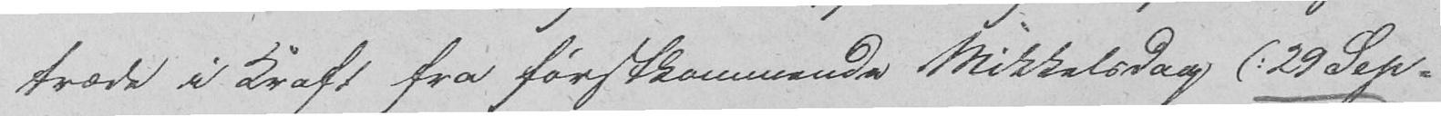træde i Kraft fra førstkommende Mikkelsdag (: 29 Sep-
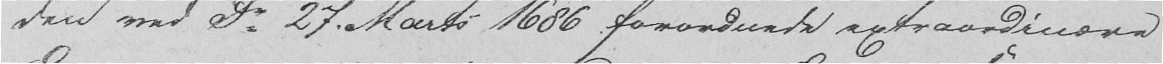den ved Fr 27 Marts 1686 forordnede extraordinære
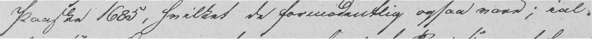Paaske 1685, hvilket de formodentlig ogsaa vare; ial-
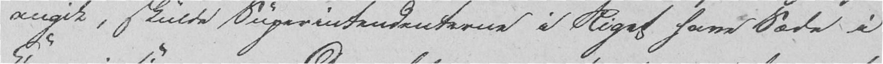angik, skulde Superintendanterne i Riget have Sæde i
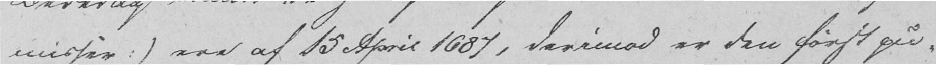misser) ere af 15 April 1687, derimod er den først pu-
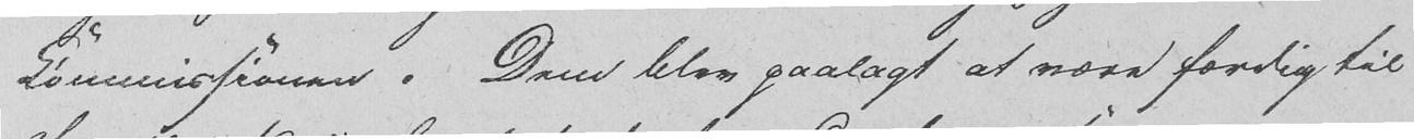Kommissionen. Dem blev paalagt at være færdig til
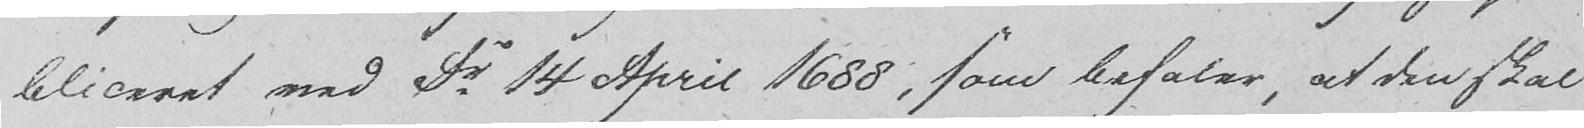bliceret ved Fr 14 April 1688, som befaler, at den skal
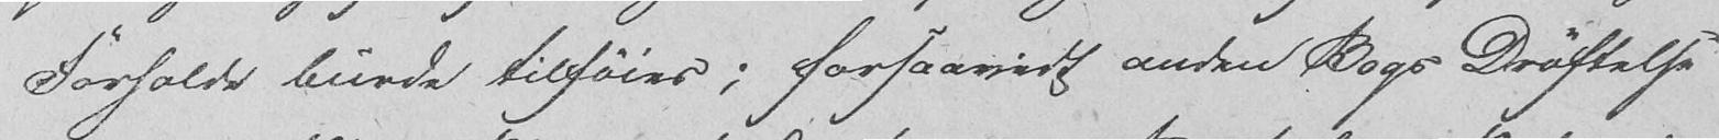Forholde burde tilføies; forsaavidt anden Bogs Drøftelse
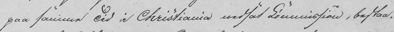paa samme Tid i Christiania nedsat Kommission, bestaa-
